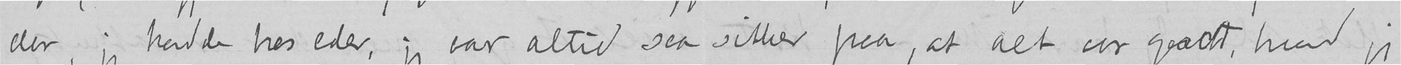der, jeg havde hos eder, jeg var altid saa sikker paa, at det var godt, hvad jeg
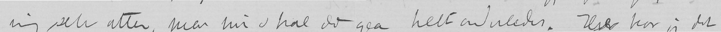mig selv atter, men nu skal det gaa helt anderledes. Her har jeg det
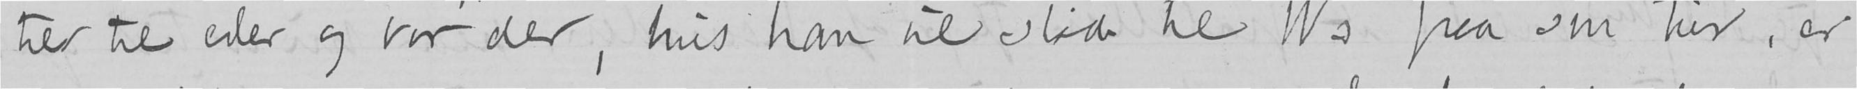ter til eder og var der, hvis han vil staa til Ws paa sin tur, er
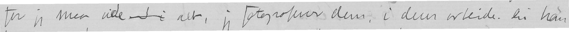for jeg maa vide end i alt, jeg fotograferer dem, i deres arbeide. Du kan
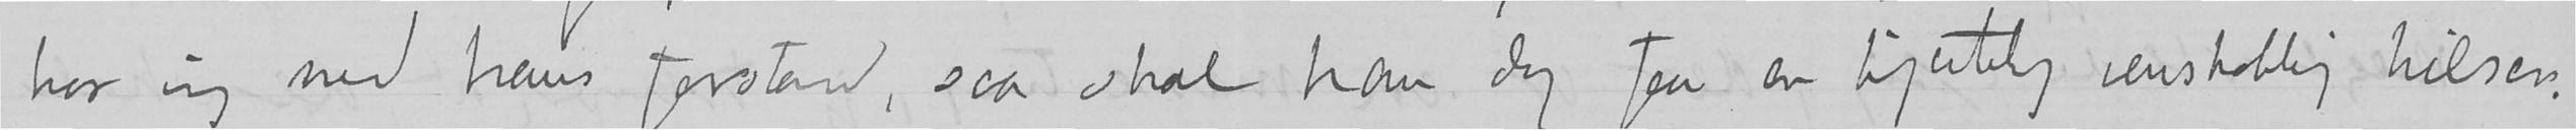har sig med hans forstand, saa skal han dog faa en hjertelig venskablig hilsen.
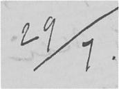29/7.
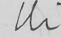bli
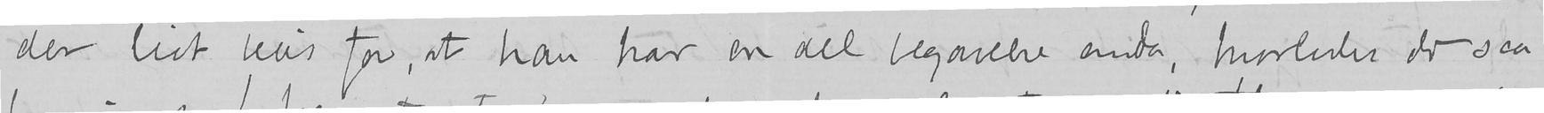der lidt bevis for, at han har en del begavelse enda, hvorledes det saa
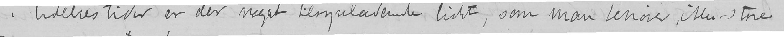i lidelsestider er der noget tilsyneladende lidet, som man behøver, ikke store
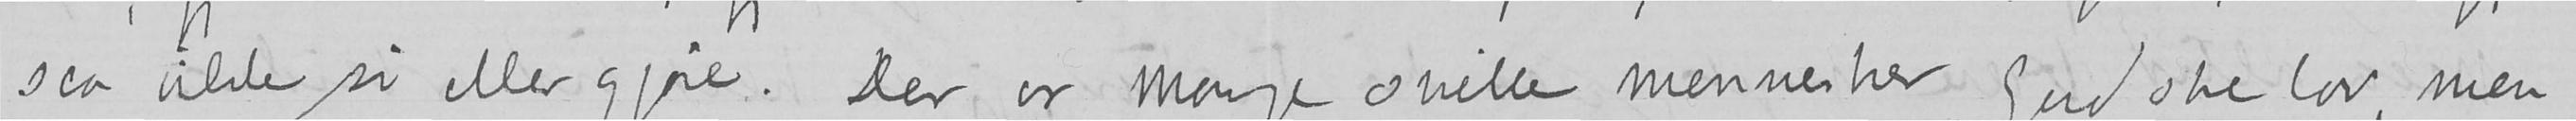saa vilde si eller gjøre. Der er mange snille mennesker, Gud ske lov, men
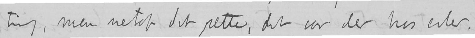ting, men netop det rette, det var der hos eder.
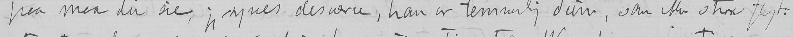paa maa du sie, jeg synes desværre, han er temmelig dum, som ikke strax flyt-
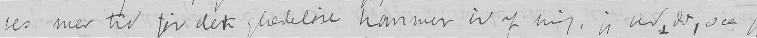ves mer tid før det glædeløse kommer ud af mig, jeg ved, det, saa jeg
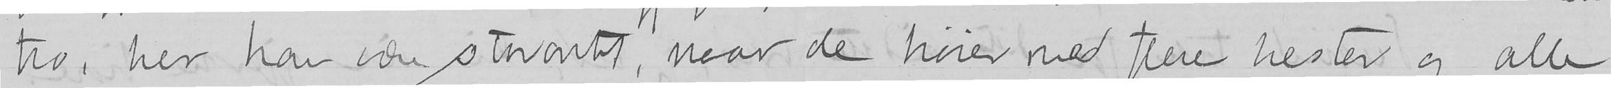tro, her har være storartet, naar de høier med flere hester og alle
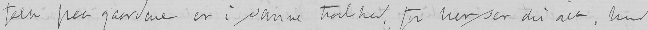folk paa gaardene er i samme travlhed, for her ser du alt, hvad
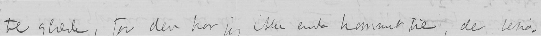til glæde, for der har jeg ikke enda kommet til, der behø-
